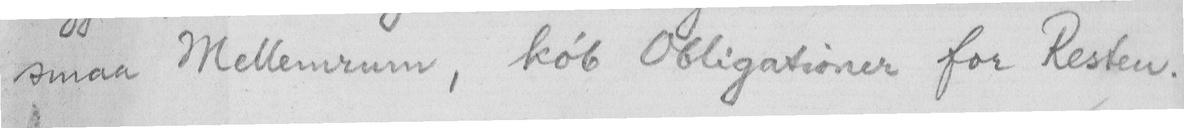smaa Mellemrum, køb Obligationer for Resten.
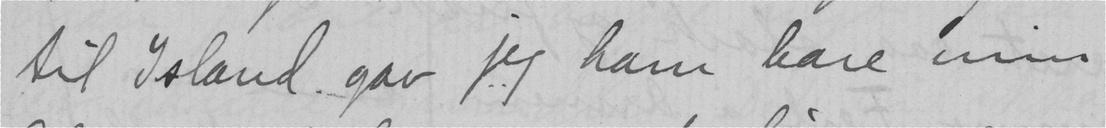til Island, gav jeg ham bare min
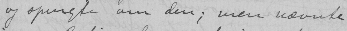og spurgte om den; men nævnte
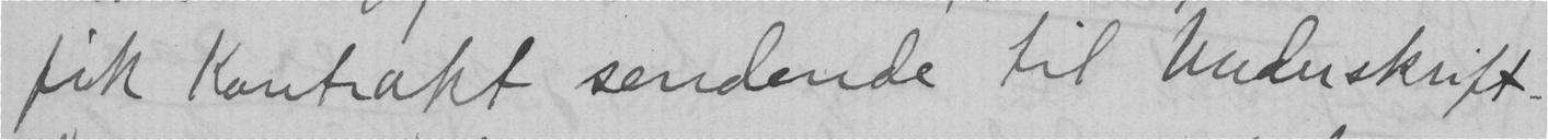fik Kontrakt sendende til underskrift.
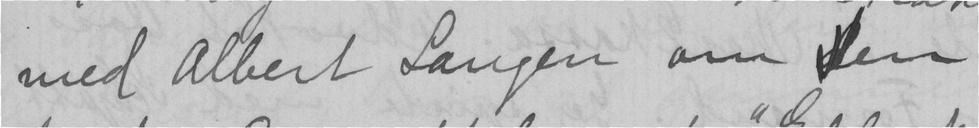med Albert Langen om en
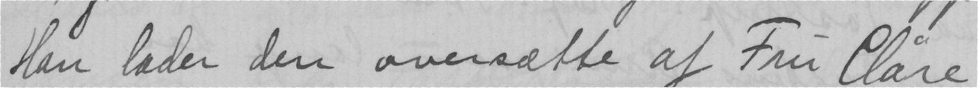Han lader den oversætte af Fru Claire
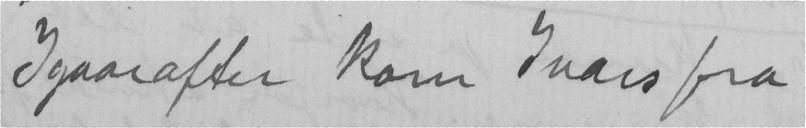Igaaraften kom Ivars fra
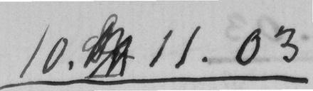10.  11.03
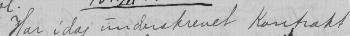Har i dag underskrevet Kontrakt
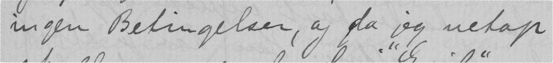ingen Betingelser, og da jeg netop
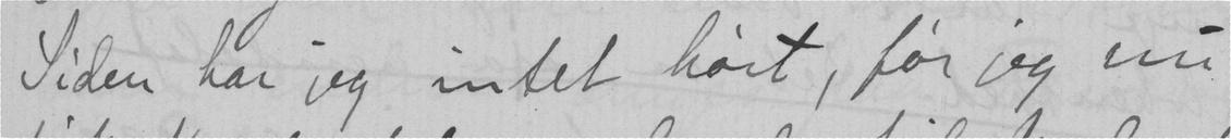Siden har jeg intet hørt, før jeg nu
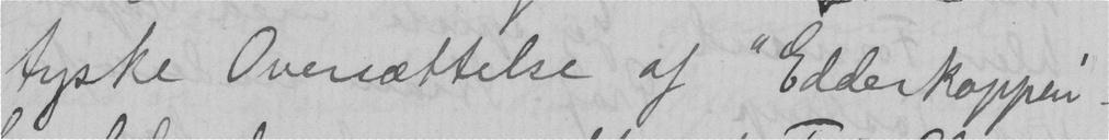tyske Oversættelse af "Edderkoppen."
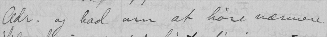adr. og bad om at høre nærmere.
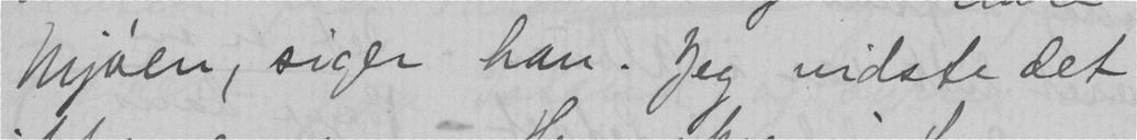Mjøen, siger han. Jeg vidste det
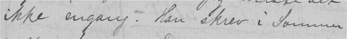ikke engang. Han skrev i Sommer
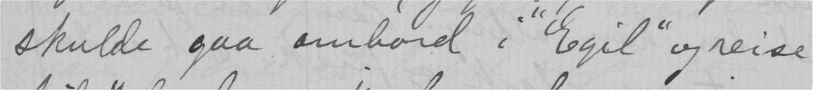skulde gaa ombord i "Egil" og reise
